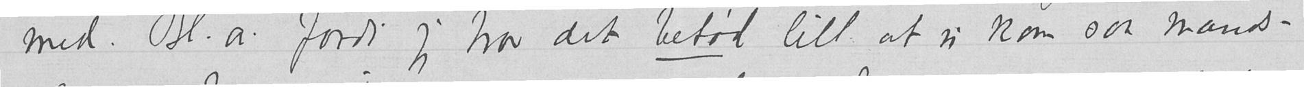med. Bl.a. fordi jeg tror det betød litt at vi kom saa mands-
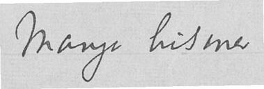Mange hilsener
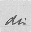din
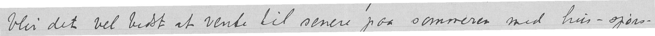blir det vel bedst at vente til senere paa sommeren med hus-spørs-
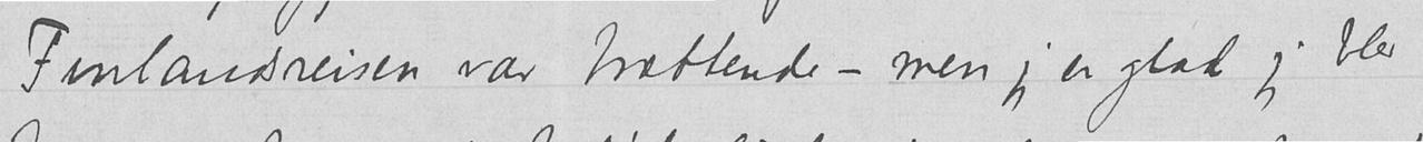Finlandsreisen var trættende – men jeg er glad jeg blev
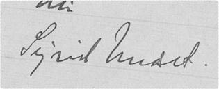Sigrid Undset.
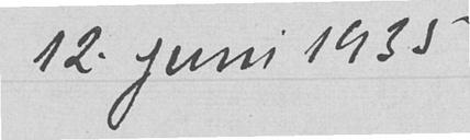12. juni 1935
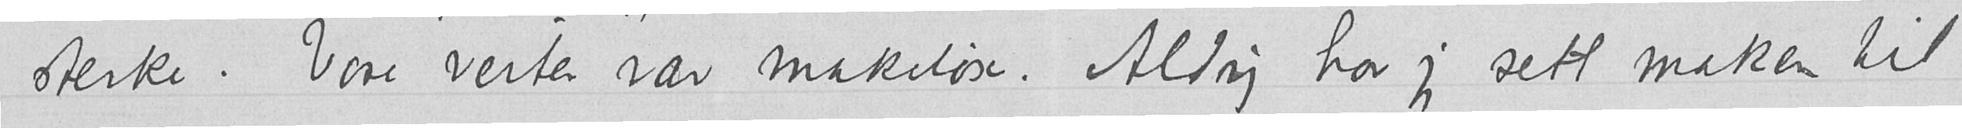sterke. Vore verter var makeløse. Aldrig har jeg sett maken til
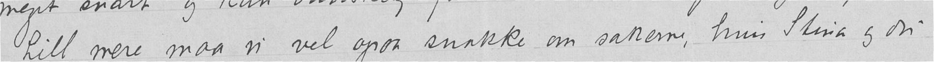Litt mere maa vi vel ogsaa snakke om sakerne, hvis Stina og du
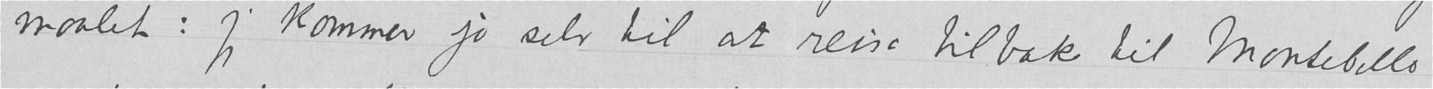maalet: jeg kommer jo selv til at reise tilbake til Montebello
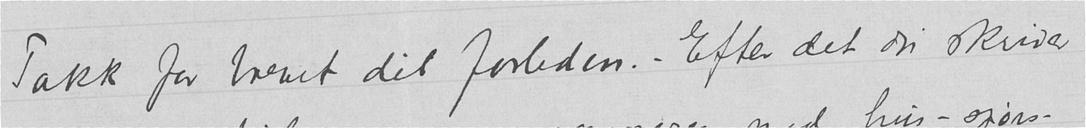Takk for brevet dit forleden. – Efter det du skriver
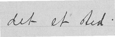det et sted.
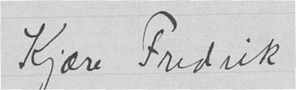Kjære Fredrik
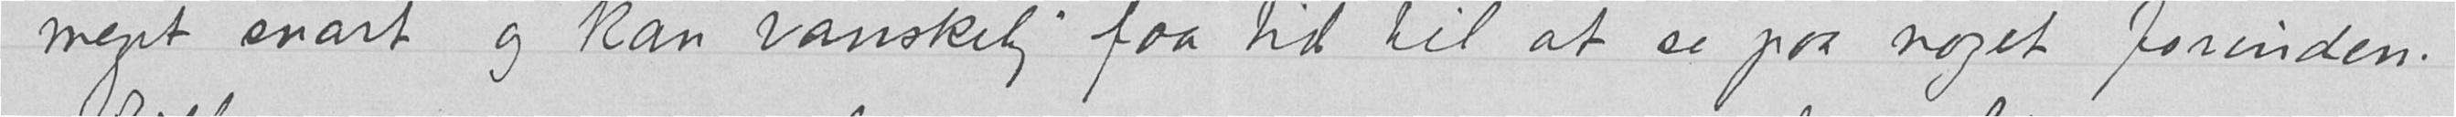meget snart og kan vanskelig faa tid til at se paa noget forinden.
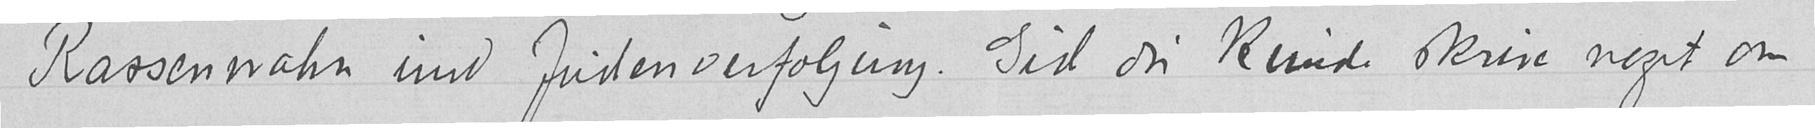Rassenwahn ind Judenverfolgung. Gid du kunde skrive noget om
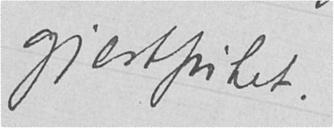gjestfrihet.
